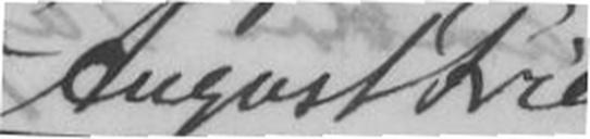August Fries
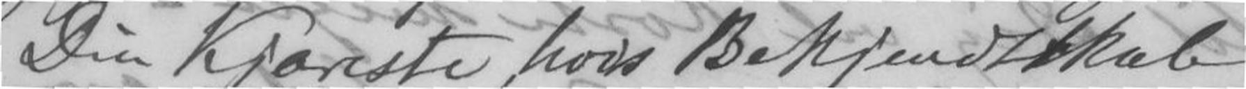Din Kjæreste hvis Bekjendtskab
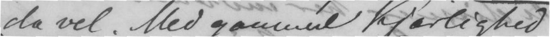da vel. Med gammel kjærlighed
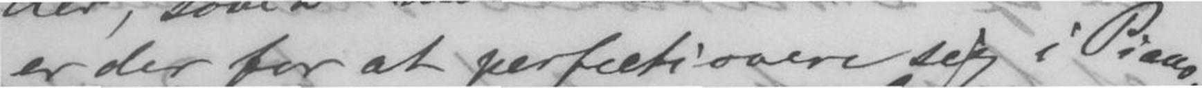er der for at perfectionere sig i Piano-
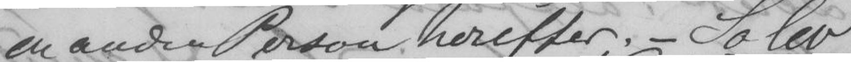en anden Person herefter. - So lev
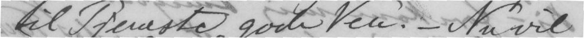til Tjeneste gode Ven. - Nu vil
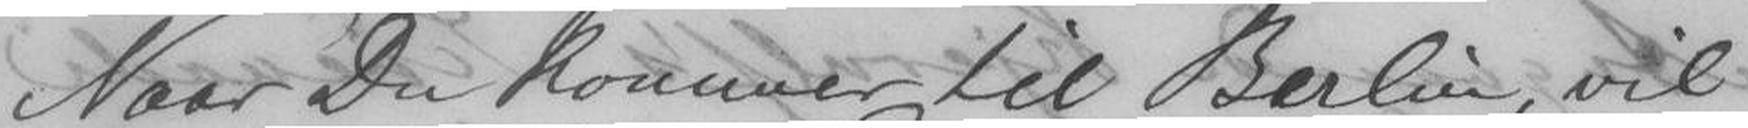Naar Du kommer til Berlin vil
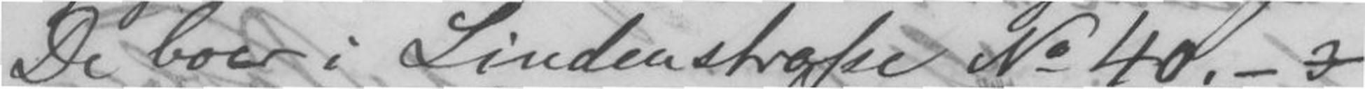De boer i Lindenstrasse No 40. -
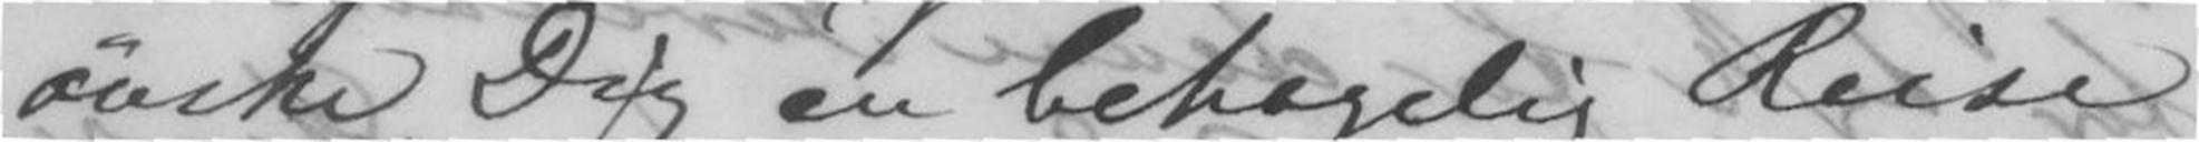ønske Dig en behagelig Reise
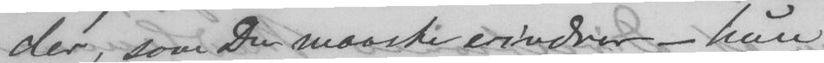der, som Du maaske erindrer - hun
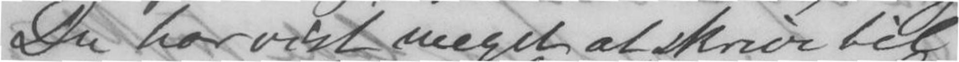Du har vist meget at skrive til
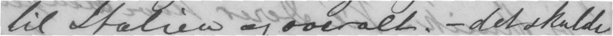til Italien og overalt; - det skulde
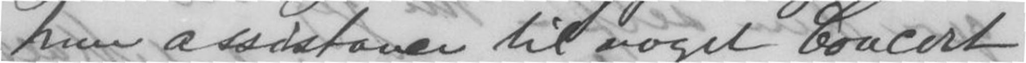hun assistance til noget Concert
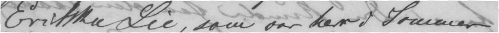Erikka Lie, som var her i Sommer
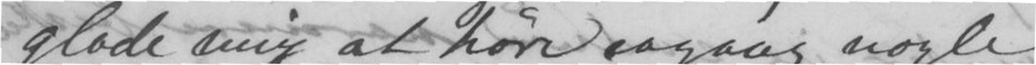glæde mig at høre engang nogle
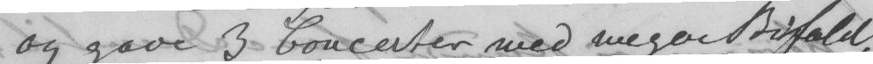og gave 3 Concerter med megen Bifald.
